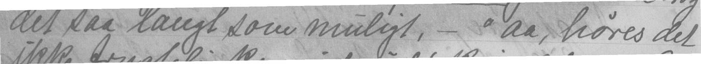det saa langt som muligt, - "aa, høres det
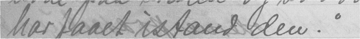har faaet istand den."
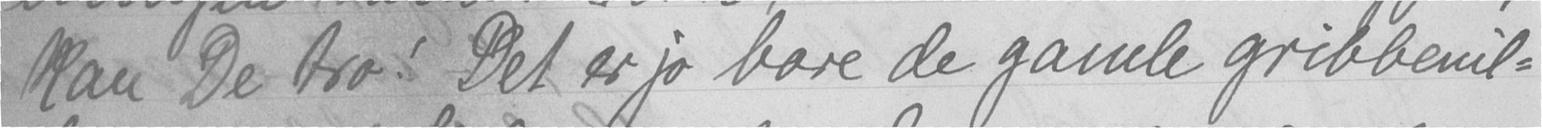kan De tro! Det er jo bare de gamle gribbenil-
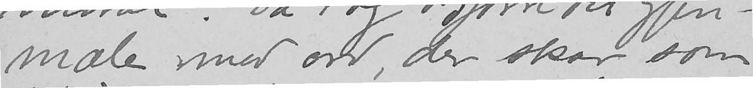mæle med ord, der skar som
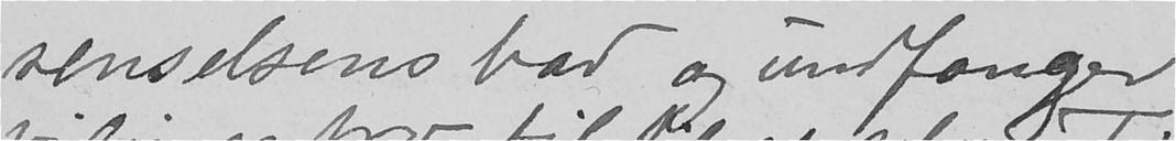renselsens bad og undfanger
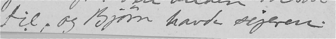til, og Bjørn havde sejeren.
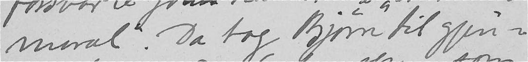moral". Da tog Bjørn til gjen-
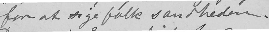for at sige folk sandheden.
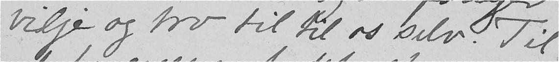vilje og tro til til os selv. Til
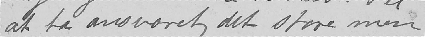at ta ansvaret, det store men
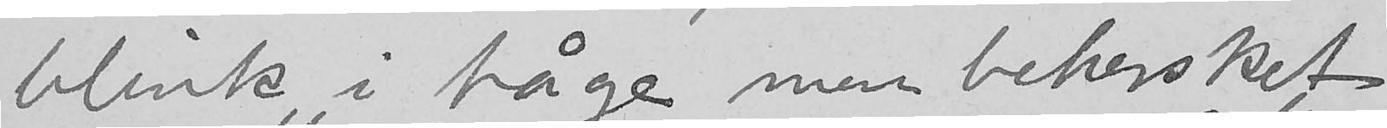blink i tåge, men behersket
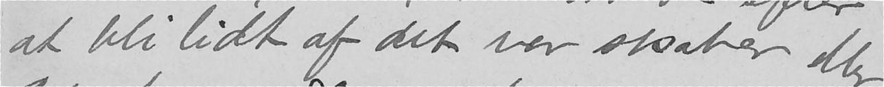at bli lidt af det vor skaber eller
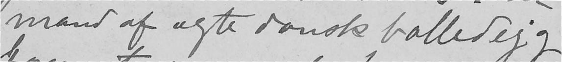mand af ægte dansk bolledejg
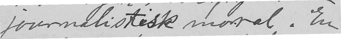journalistisk moral. En
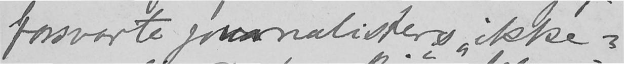forsvarte journalisters, "ikke-
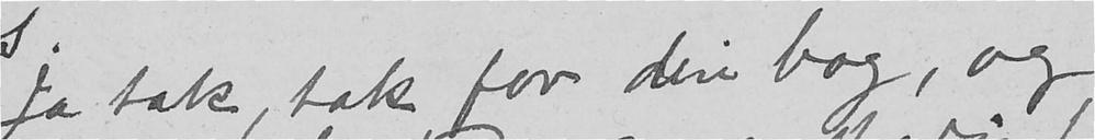Ja tak, tak for din bog, og
